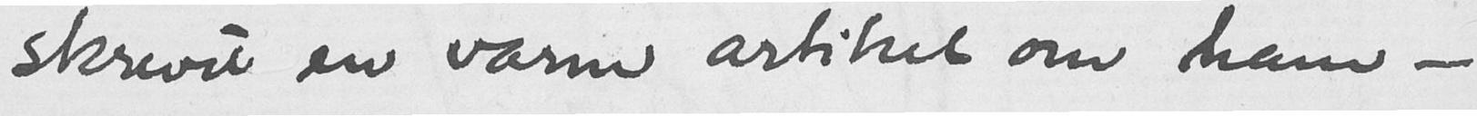skrevet en varm artikel om ham -
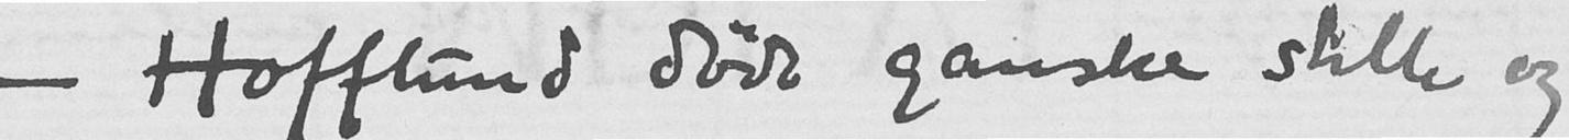- Hofflund døde ganske stille og
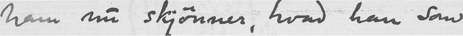ham nu skjønner, hvad han som
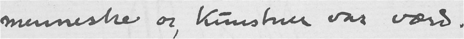menneske og kunstner var værd.
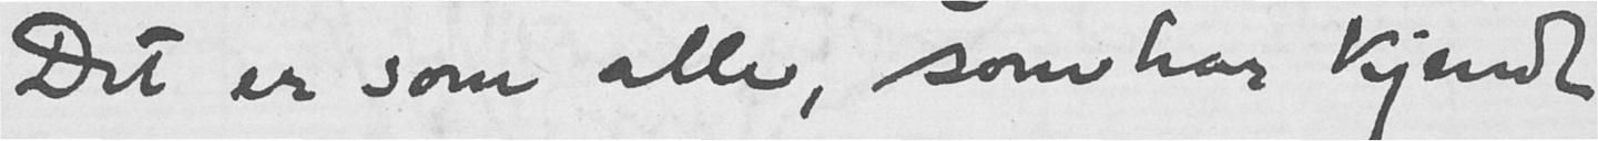Det er som alle, som har kjendt
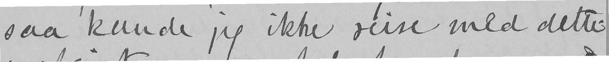saa kunde jeg ikke reise med dette
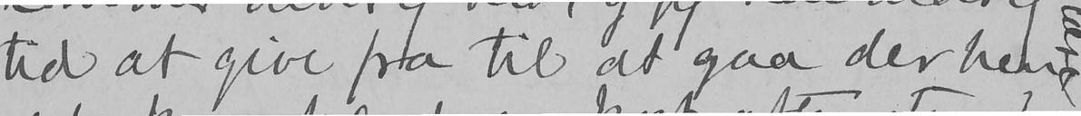tid at give fra til at gaa derhen,
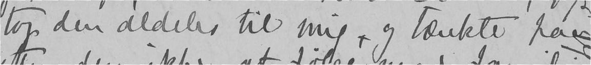tog den aldeles til mig, og tænkte paa
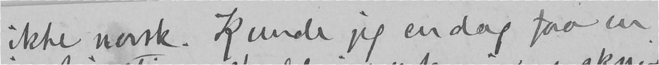ikke norsk. Kunde jeg en dag faa en
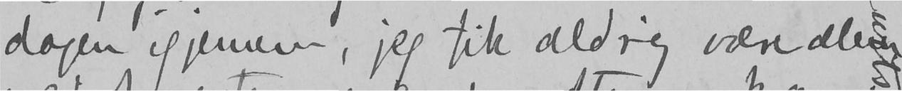dagen igjennem, jeg fik aldrig være alene
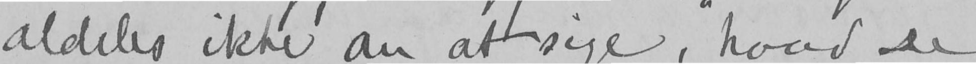aldeles ikke an at sige, hvad De
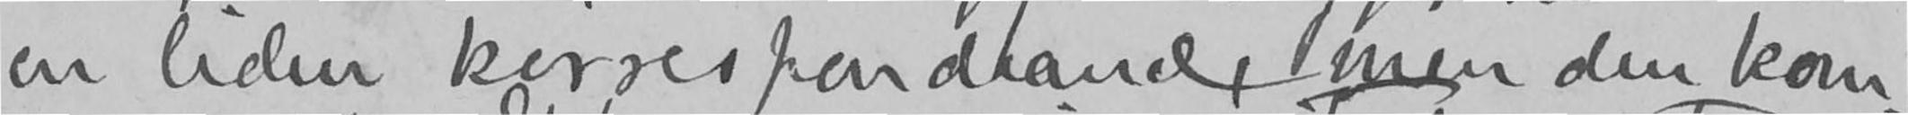en liden korrespondance, men den kom-
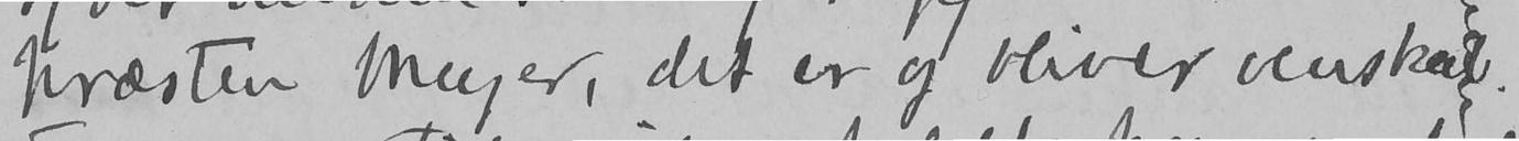præsten Meyer, det er og bliver venskab.
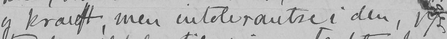og kraft, men intolerantse i den, jeg
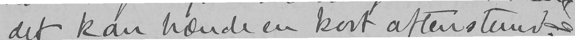det kan hænde en kort aftenstund.
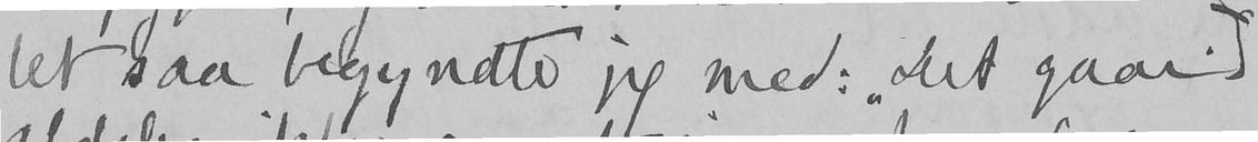let saa begyndte jeg med: "Det gaar
